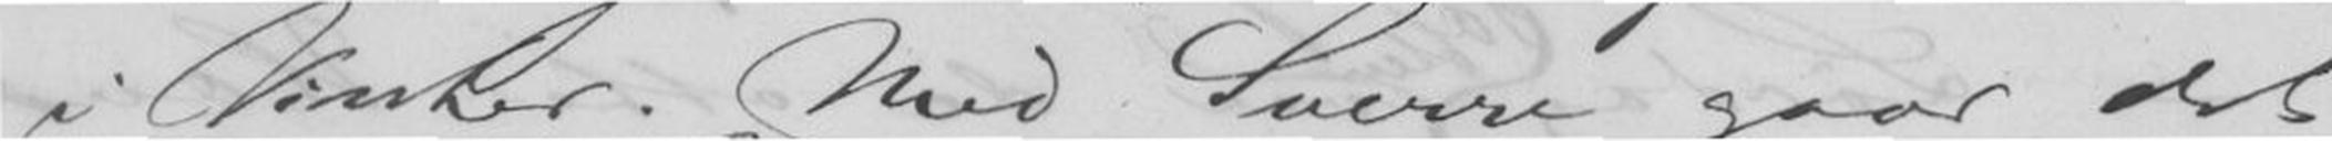i Vinter. Med Sverre gaar det
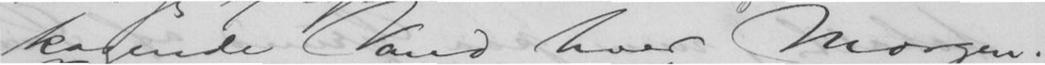kogende Vand hver Morgen.
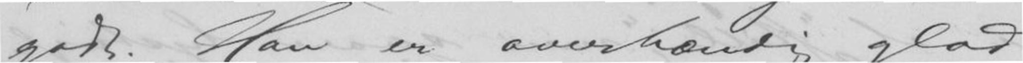godt. Han er overhændig glad
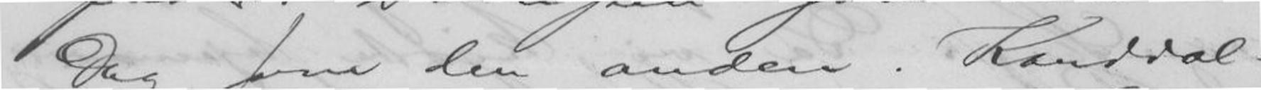Dag som den anden. Kardial-
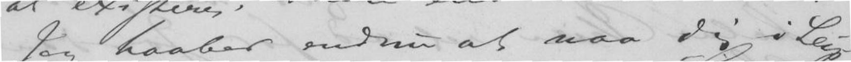Jeg haaber endnu at naa Dig i Leip-
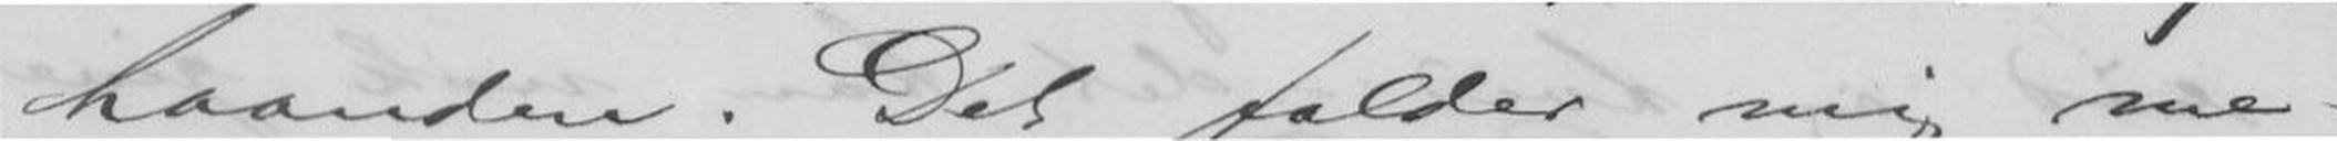haanden. Det falder mig me-
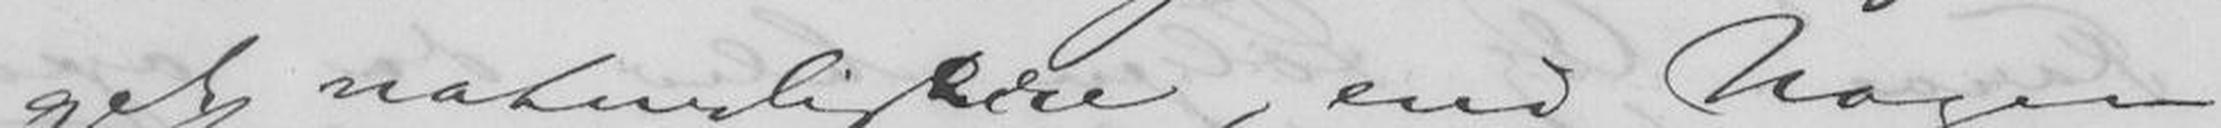get naturligere, end Nogen
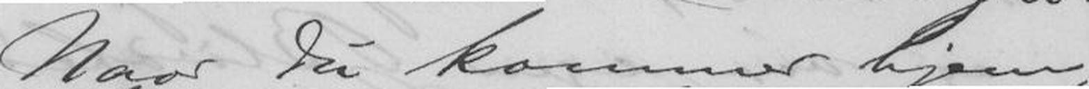Naar Du kommer hjem,
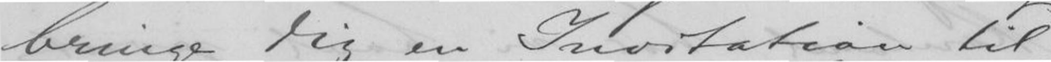bringe dig en Invitation til
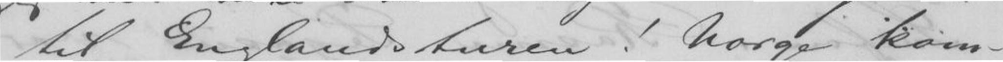til Englandsturen! Norge kom-
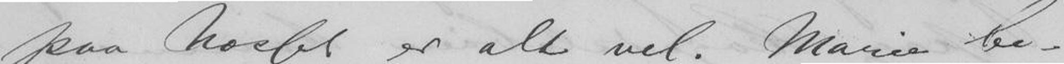paa Næsset er alt vel. Marie be-
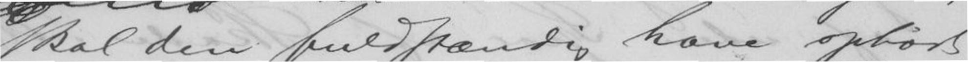skal den fuldstændig have ophørt
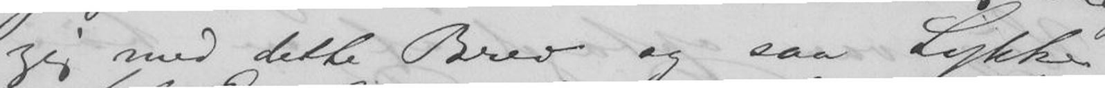zig med dette Brev og saa Lykke
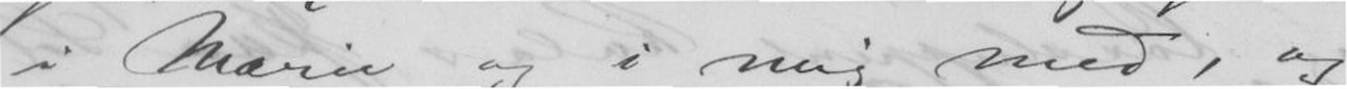i Marie og i mig med, og
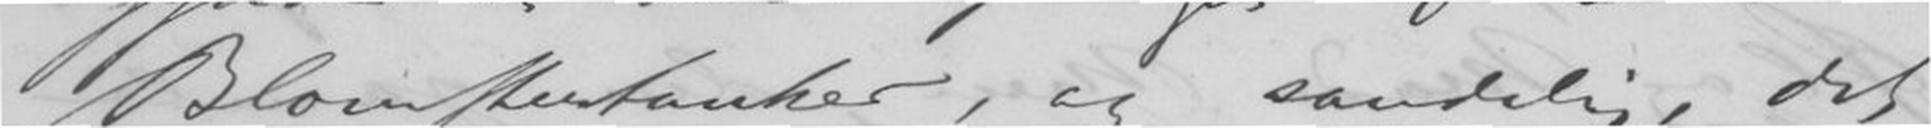Blomstertanker, og sandelig, det
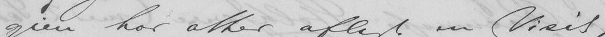gien har atter aflagt en Visit,
